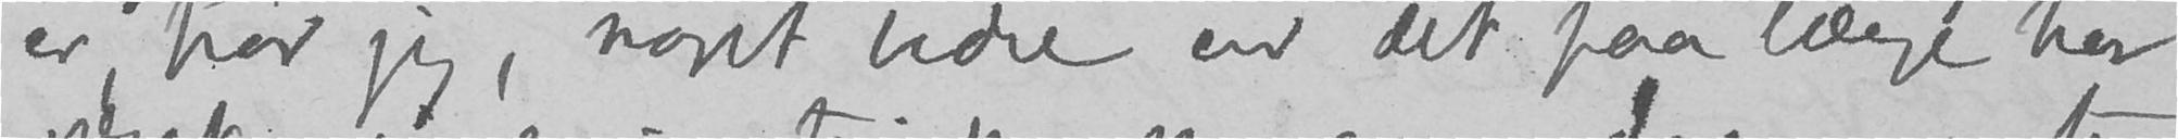er, tror jeg, noget bedre end det paa længe har
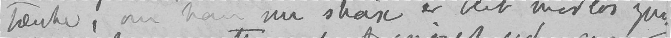tænke, om han nu strax er blit modløs igjen.
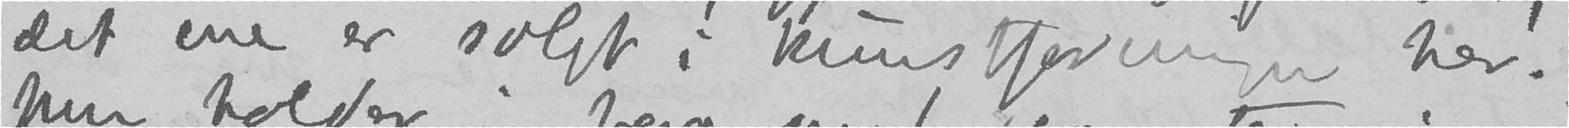det ene er solgt i kunstforeningen her.
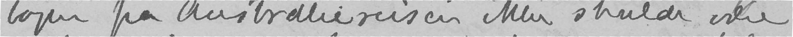bogen fra Australiereisen ikke skulde være
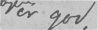er god,
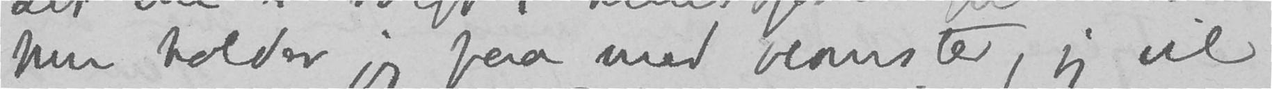Nu holder jeg paa med de næste, jeg vil
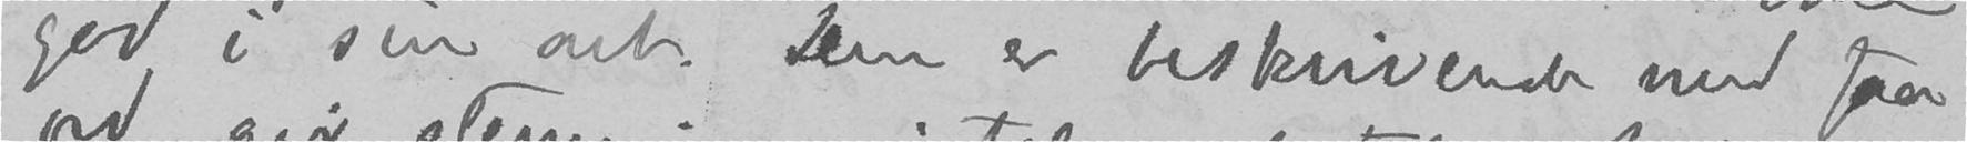god i sin art. Den er beskrivende med faa
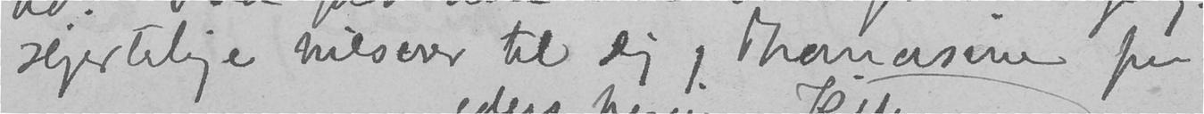Hjertelige hilsener til Dig og Thomasine fra
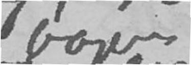bogen
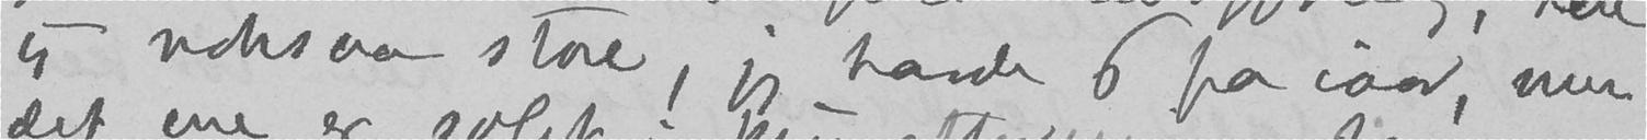og noksaa store, jeg havde 6 fra iaar, men
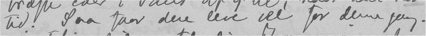tid. Saa faar dere leve vel for denne gang.
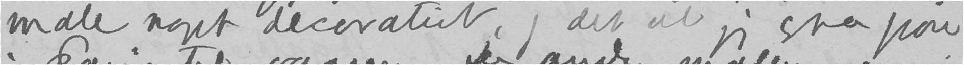male noget decorativt, det vil jeg ogsa gjøre
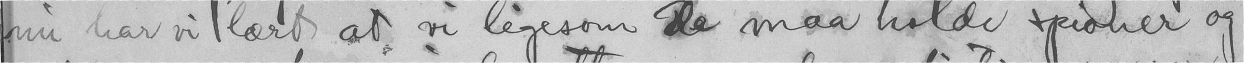nu har vi lært at vi ligesom de maa holde spioner og
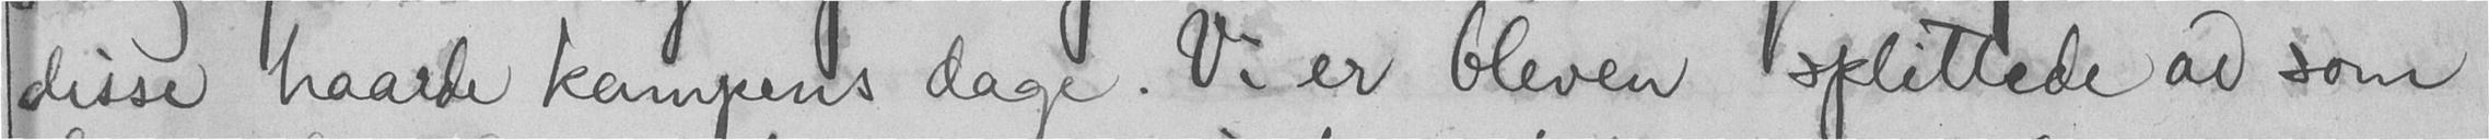disse haarde kampens dage. Vi er bleven splittede ad som
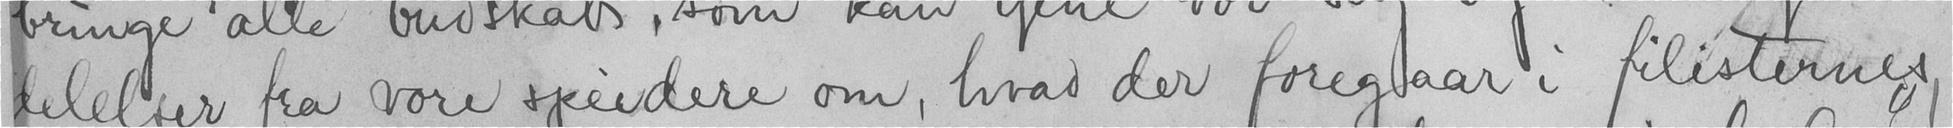delelser fra vore speidere om, hvad der foregaar i filisternes
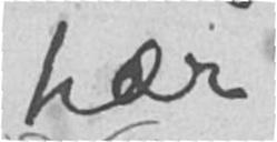hær
